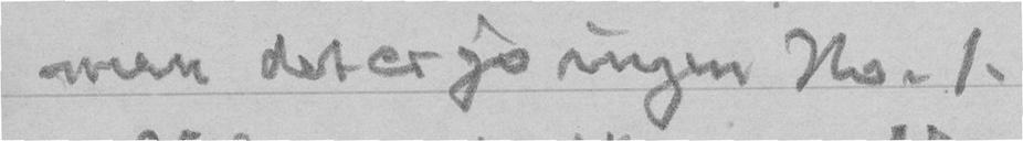men det er jo ingen No.1.
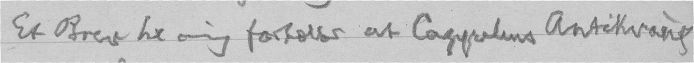Et Brev til mig fortæller at Cappelens Antikvariat
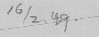16/2. 49.
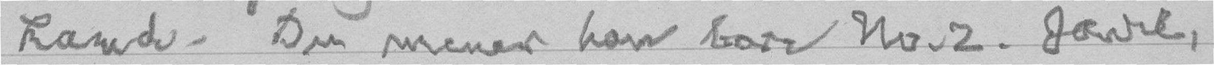Lande. Du mener han bare No.2. Javel,
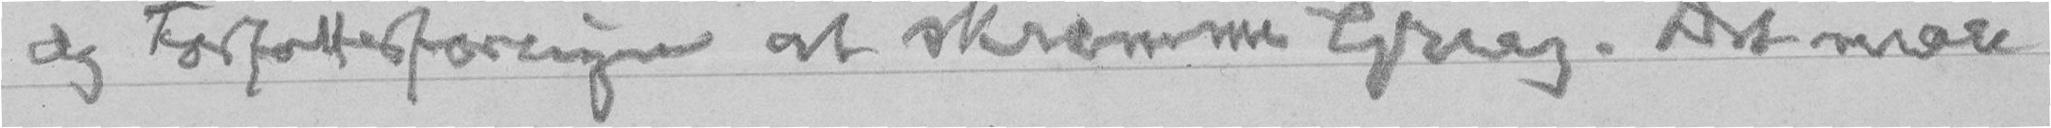og Forfatterforeningen at skræmme Grieg. Det maa
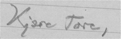Kjære Tore,
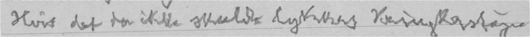Hvis det da ikke skulde lykkes Kringkastingen
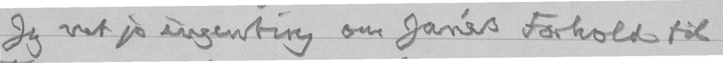Jeg vet jo ingenting om Janés Forhold til
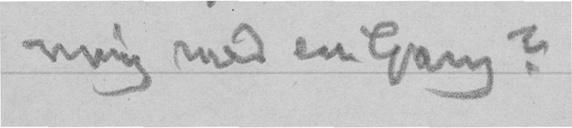mig med en Gang?
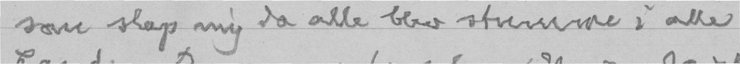som slap mig da alle blev stumme i alle
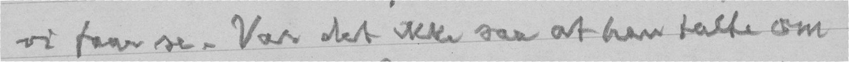vi faar se. Var det ikke saa at han talte om
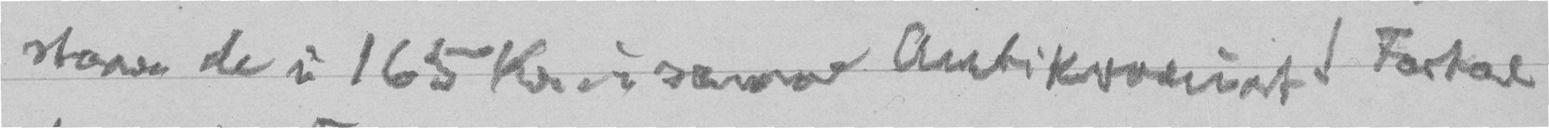staar de i 165 Kr. i samme Antikvariat! Fortæl
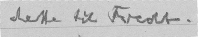dette til Tvedt.
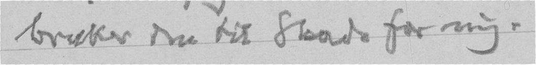bruker den til Skade for mig.
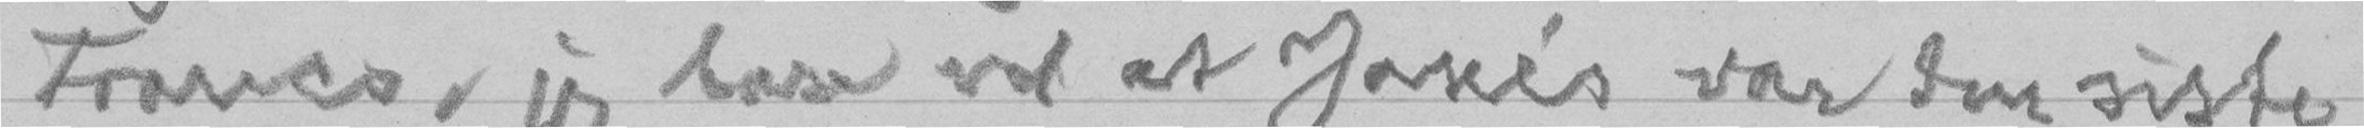Franco, jeg bare vet at Janés var den siste
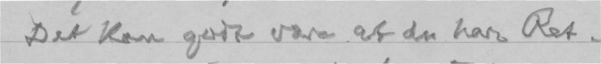Det kan godt være at du har Ret.
